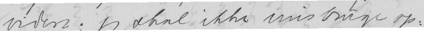videre; jeg skal ikke misbruge op-
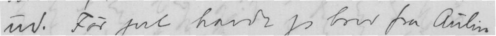ud. Før jul havde jeg brev fra Aulin
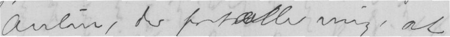Aulin, der fortæller mig, at
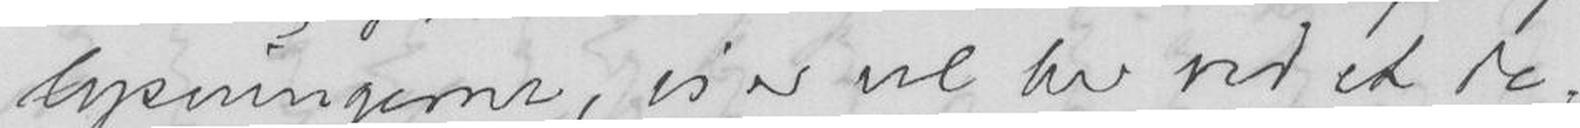lysningene, vi er vel her ved et de
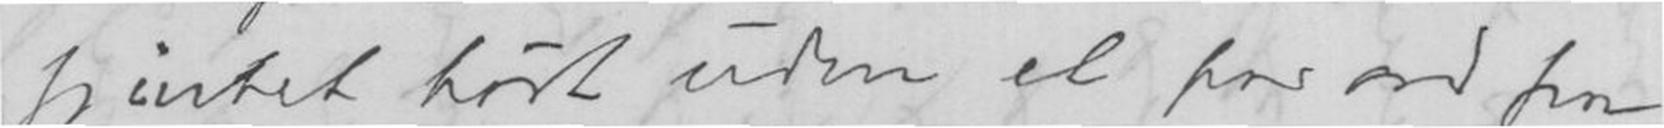jeg intet hørt uden et par ord fra
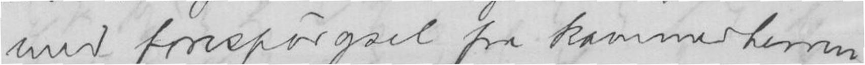med forespørgsel fra kammerherren,
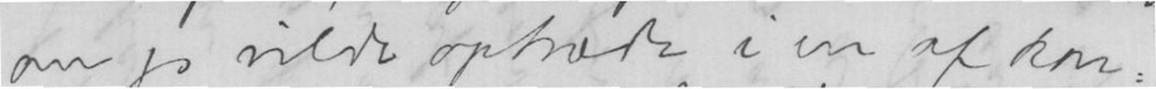om jeg vilde optræde i en af kon-
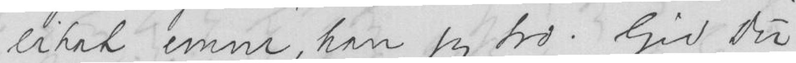likat emne, kan jeg tro. Gid Du
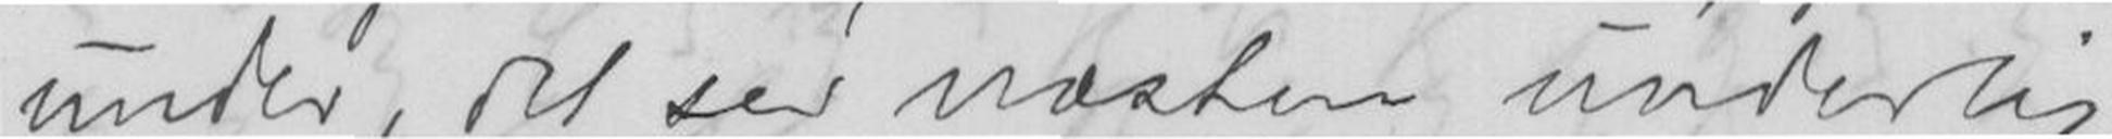under, det ser næsten underlig
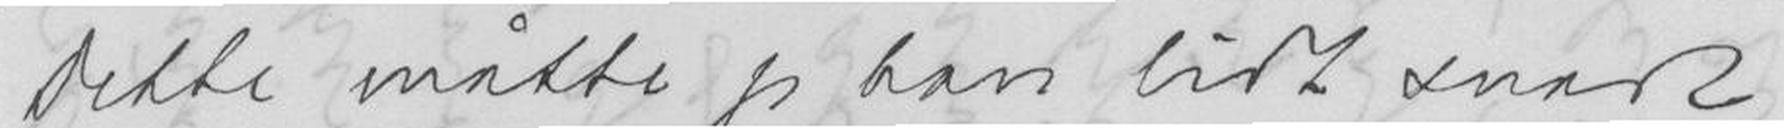Dette måtte jeg have lidt snart
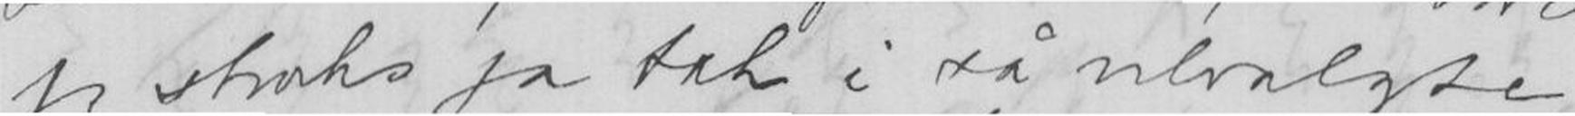jeg straks ja tak i så utvalgte
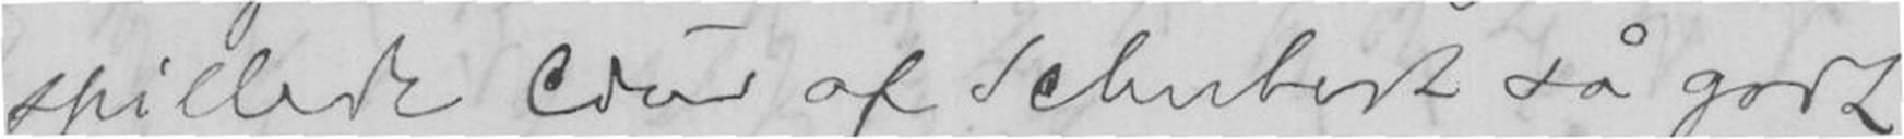spillede Cdur af Schubert så godt
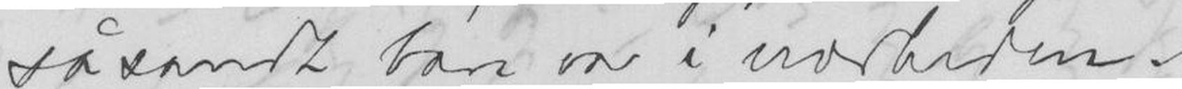såsandt bare var i nærheden. -
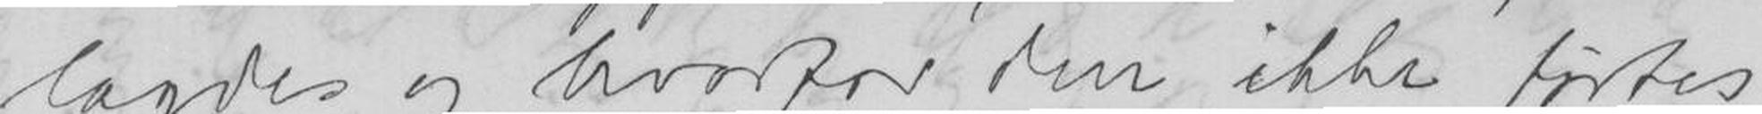lagdes og hvorfor den ikke førtes
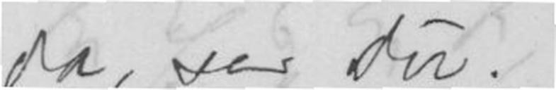da, ser du.-
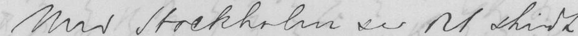Med Stockholm ser det shidt
